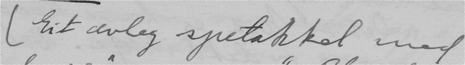(Eit avleg spetakkel med
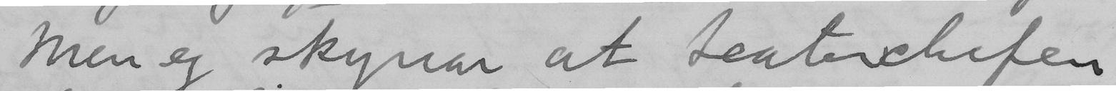Men eg skynar at teaterkafen
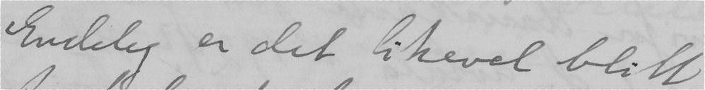Endeleg er det likevel blitt
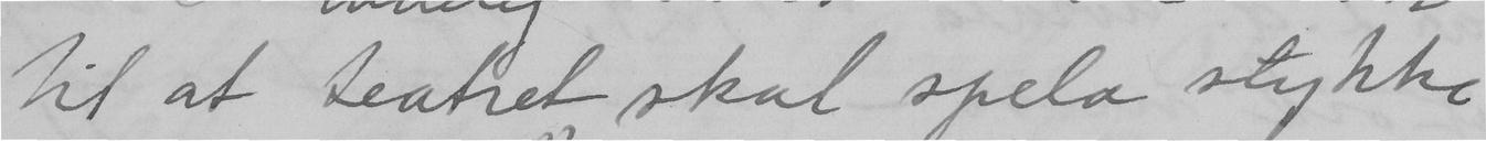til at teatret skal spela stykke
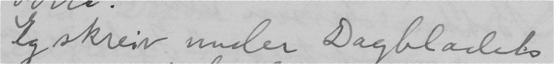Eg skreiv under Dagbladets
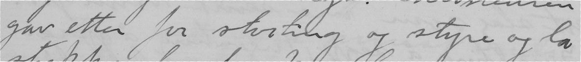gav etter for storting og styre og la
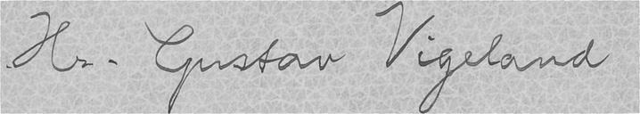Hr. Gustav Vigeland
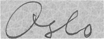Oslo
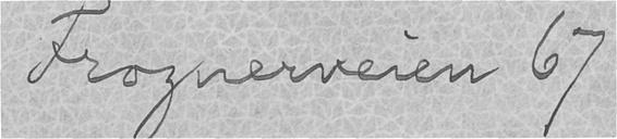Frognerveien 67
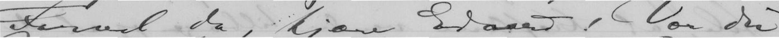Farvel da, kjære Edvard! Vær Du
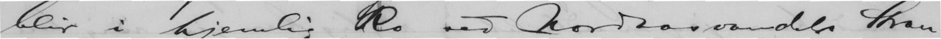blir i hjemlig Ro ved Nordaasvandets Stran-
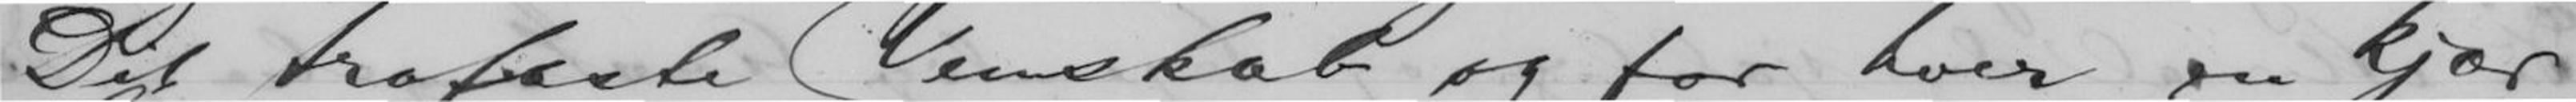Dit trofaste Venskab og for hver en kjær-
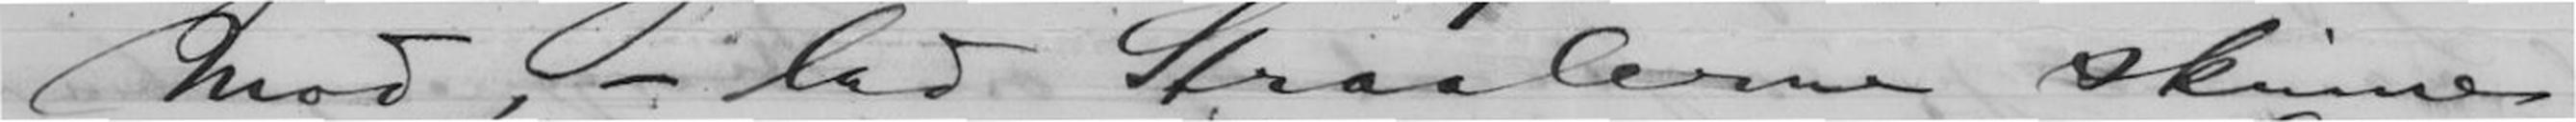Mod, - lad Straalerne skinne
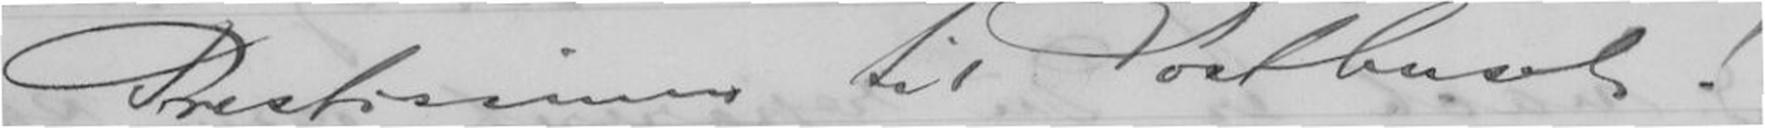Prestissimo til Posthuset!
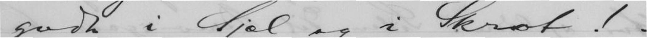godt i Sjæl og i Skrot!
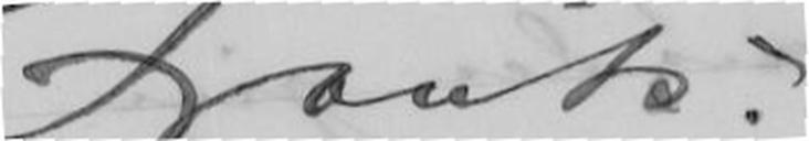Frants.
